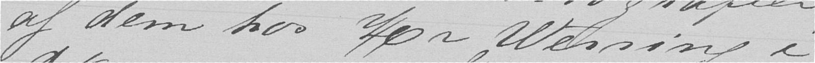af dem hos Hr Werring i
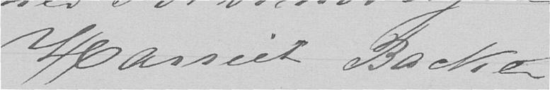Harriet Backer
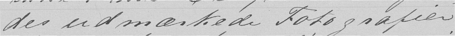des udmærkede Fotografier
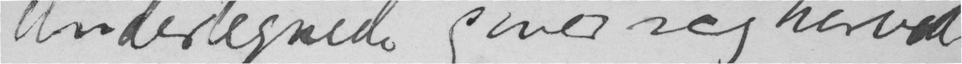Undertegnede giver sig herved
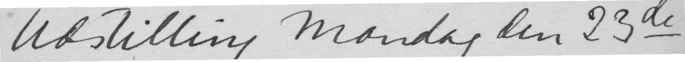Udstilling Mandag den 23de
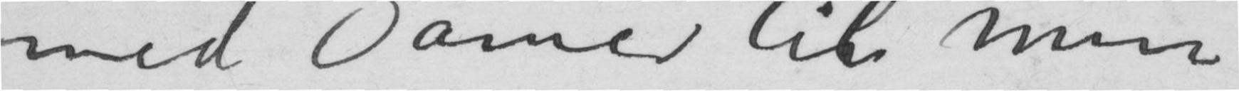med Damer til min
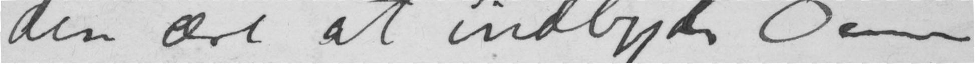den ære at indbyde Dem
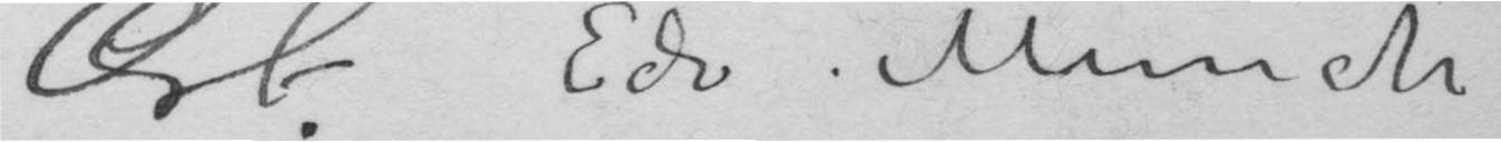Ærb. Edv. Munch
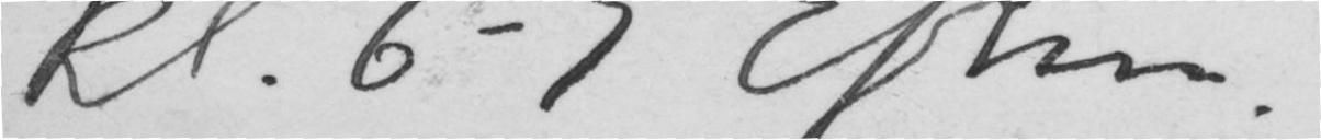Kl. 6–9 Eftm.
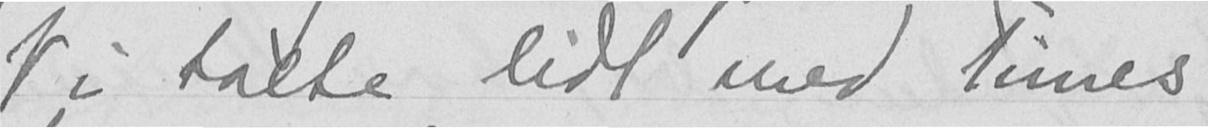Vi talte lidt med Times'
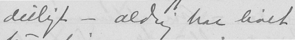deiligt - aldrig har livet
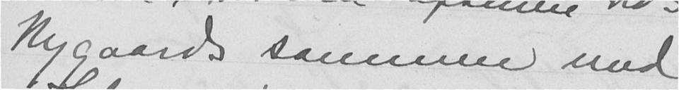Nygaards sammen med
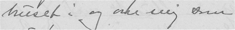bruset i og om mig som
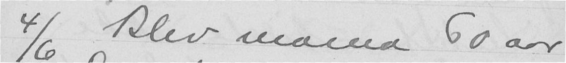4/6 Blev mama 60 aar.
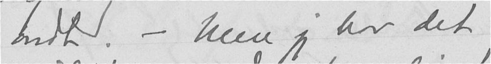ondt. - Men jeg har det
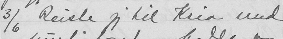3/6 Reiste jeg til Kria med
